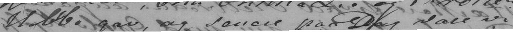Hebbe gav, og senere paa Dag vare vi
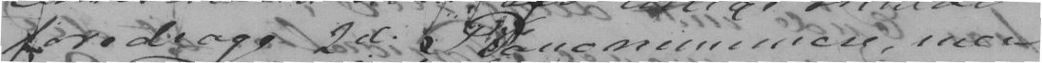foredrage 2d Pianonummere, men
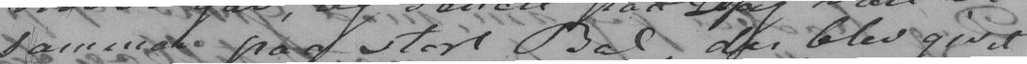sammen paa stort Bal, der blev givet
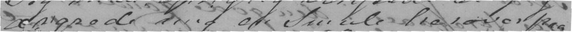angrede mig en Smule herover paa
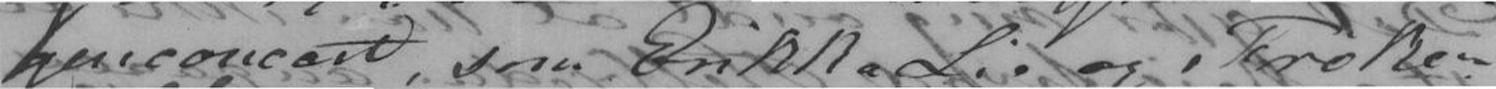genconcert, som Erikka Lie og Frøken
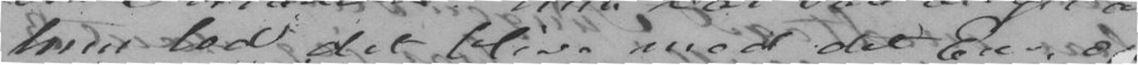hun lod det blive med det Ene, og
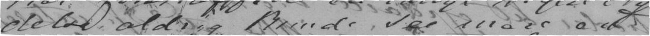delse aldrig kunde see mere end
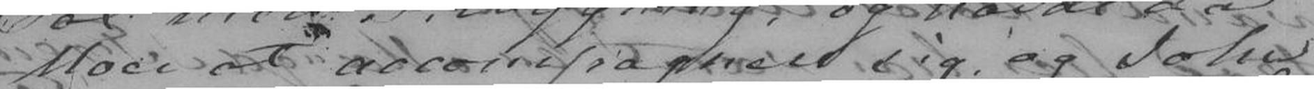Moer at accompagnere sig, og John
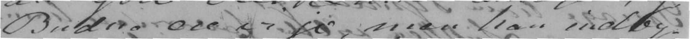Budne ere vi jo, men han indby-
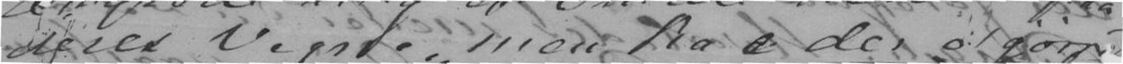deres Vegne, men ka æ der atgørre.
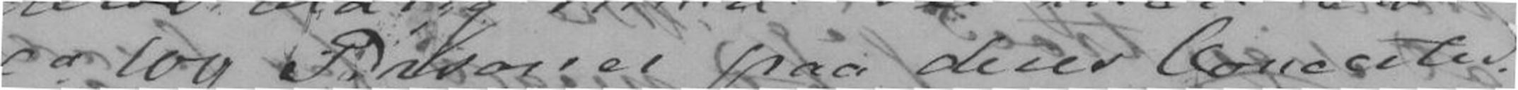ca. 100 Personer paa deres Concerter.
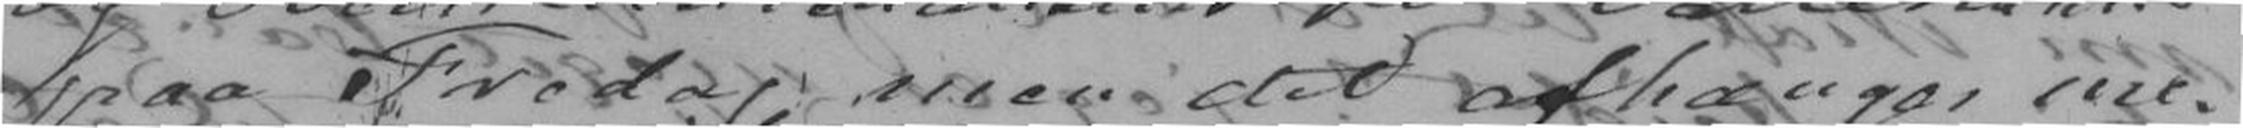paa Fredag, men det afhenger me-
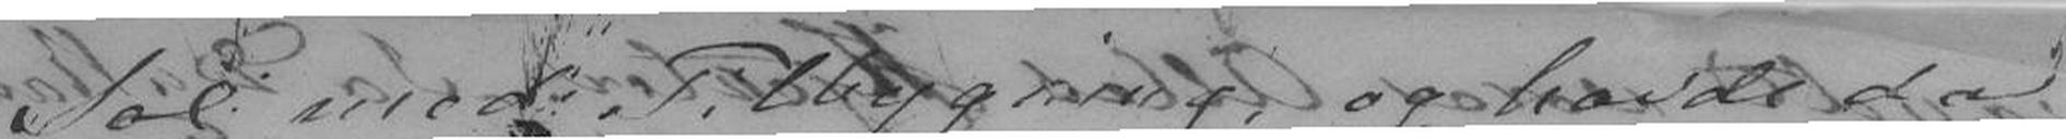Sal med Tilbygning, og havde da
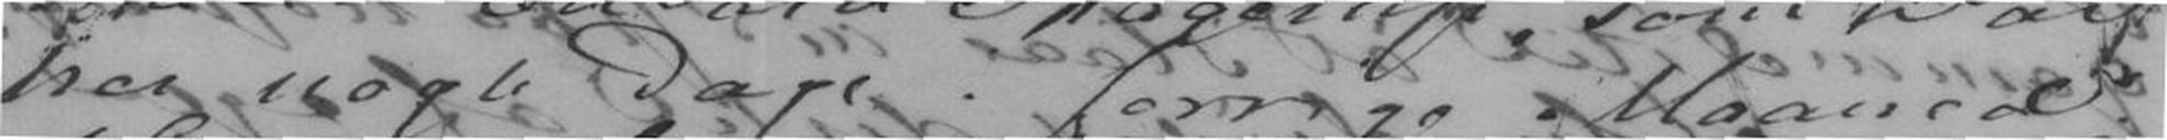her nogle Dage i forrige Maaned.
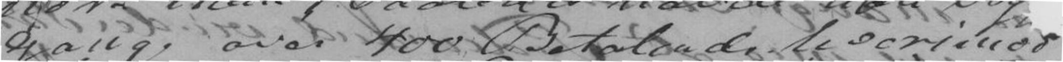Gange over 400 Betalende hvorimod
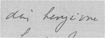din hengivne
